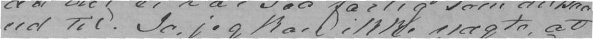ud til. Ja, jeg kan ikke nægte at
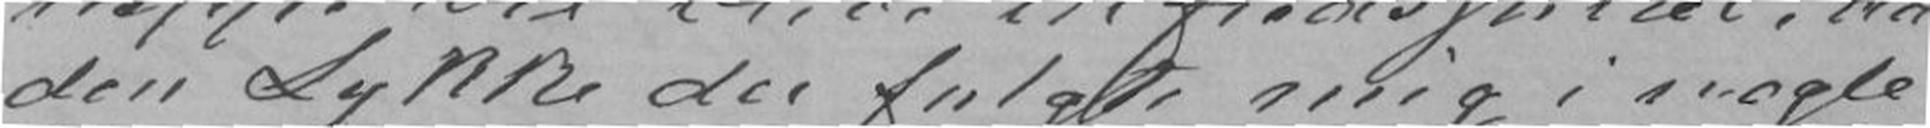den Lykke der fulgte mig i nogle
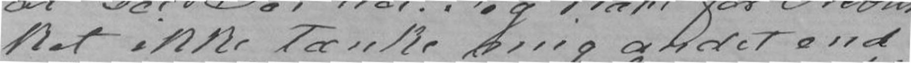ket ikke tænke mig andet end
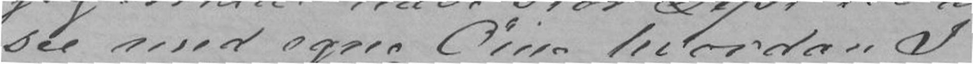see med egne Øine hvordan I
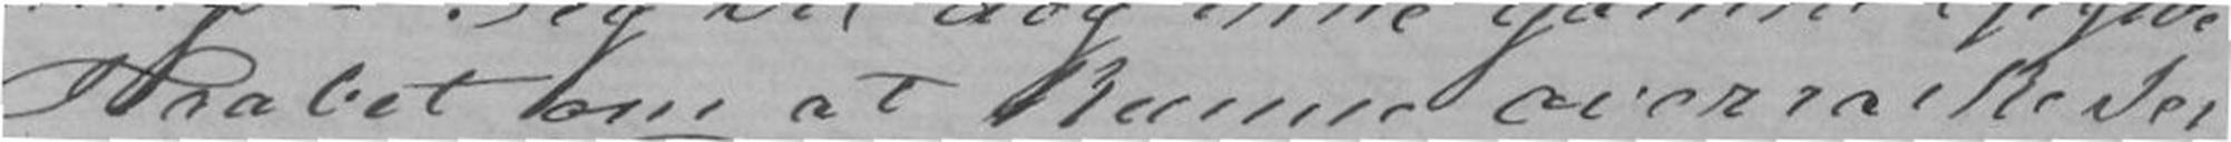Haabet om at kunne overraske Jer
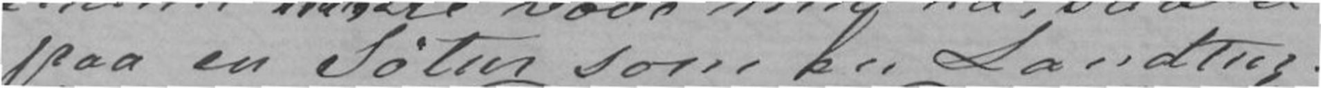paa en Søtur som en Landtur.
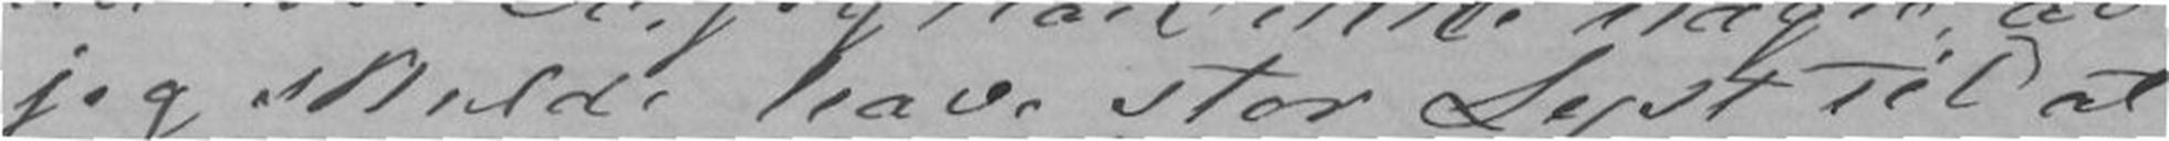jeg skulde have stor Lyst til at
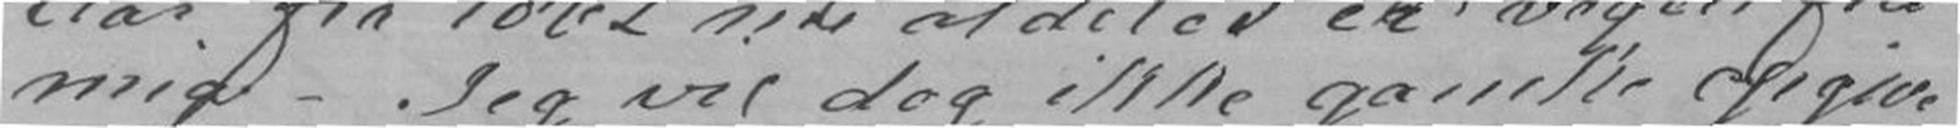mig. - Jeg vil dog ikke ganske opgive
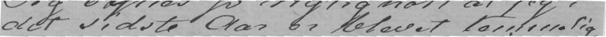det sidste Aar er blevet temmelig
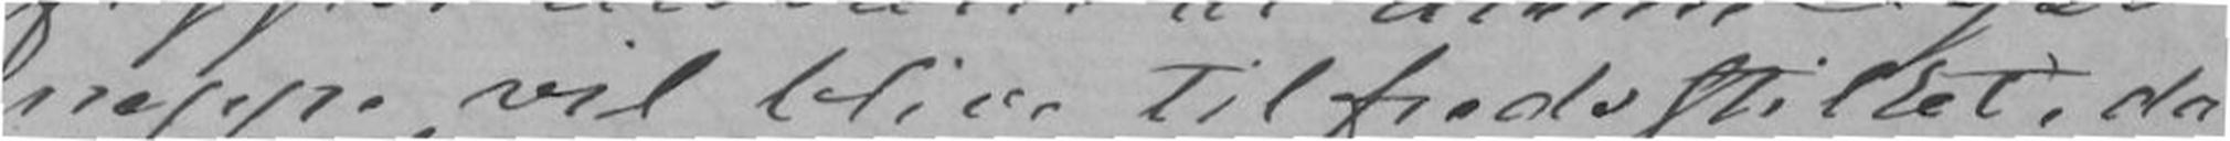neppe vil blive tilfredsstillet, da
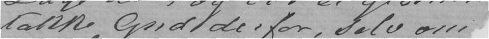takke Gud derfor, selv om
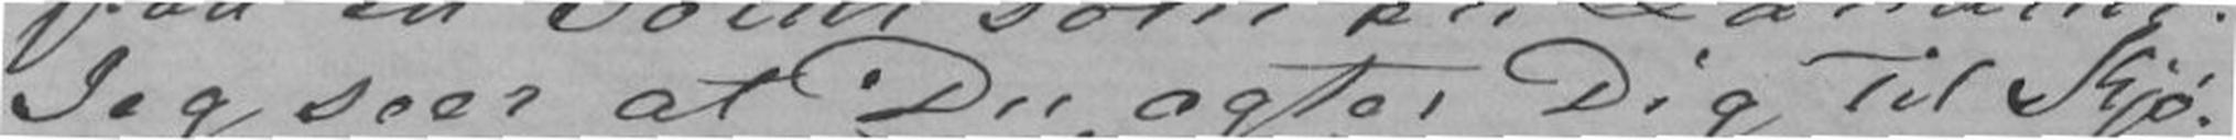Jeg seer at Du agter Dig til Kjø-
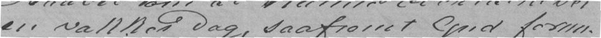en vakker Dag, saafremt Gud forun-
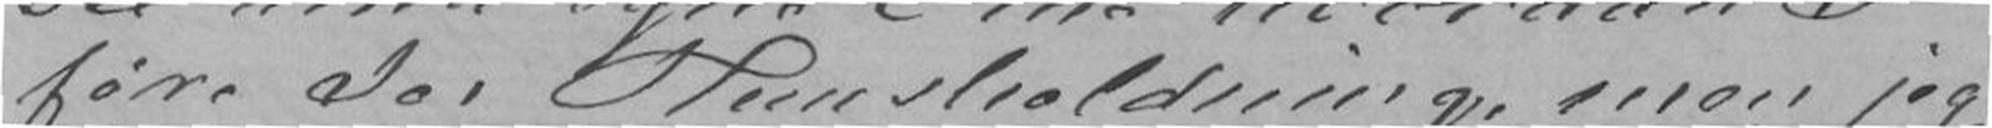føre Jer Huusholdning, men jeg
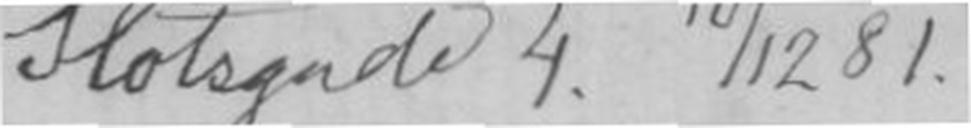Slotsgade 4. 10/12 81.
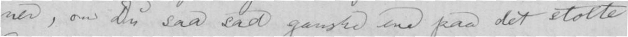ner, om Du saa sad ganske ene paa det stolte
